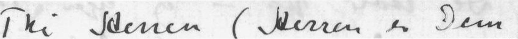Thi Herren (Herren er Dem)
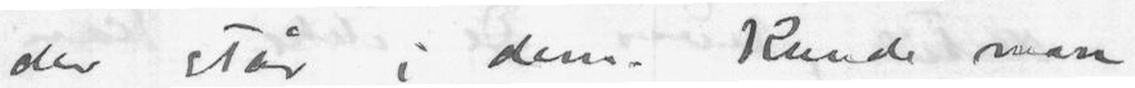der står i dem. Kunde man
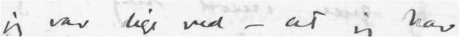jeg var lige ved - at jeg har
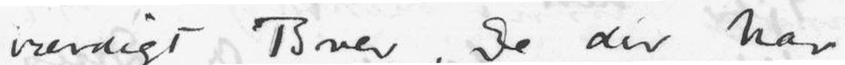værdigt Brev, De der har
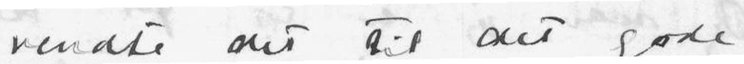vendte det til det gode.
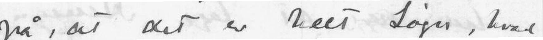på, at det er helt Løgn, hvad
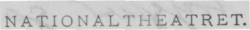NATIONALTHEATRET.
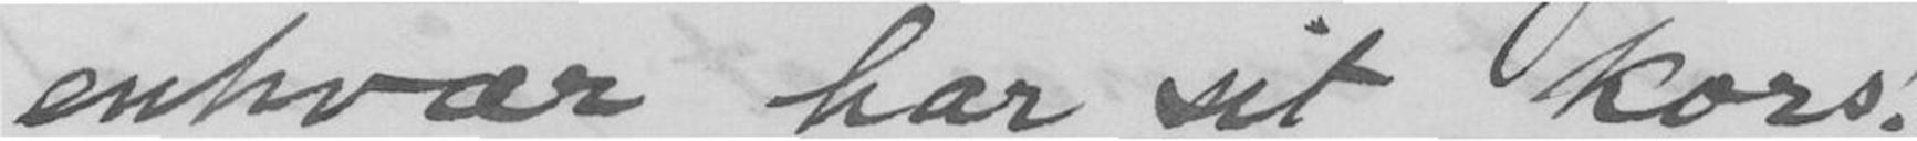enhvær har sit kors !
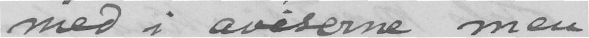med i aviserne men
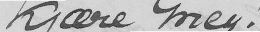Kjære Grieg !
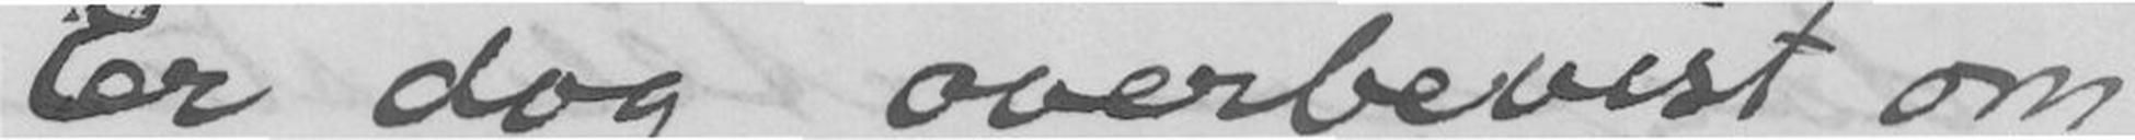Er dog overbevist om
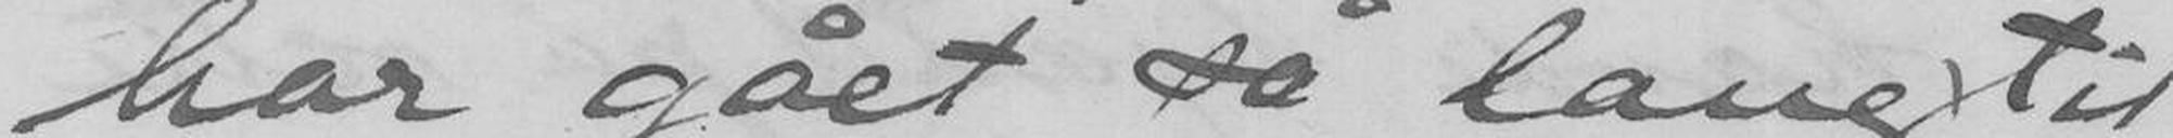har gået så lang tid
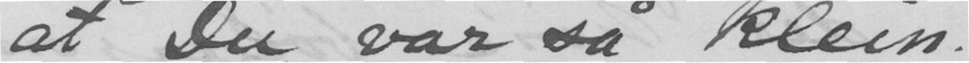at Du var så klein.
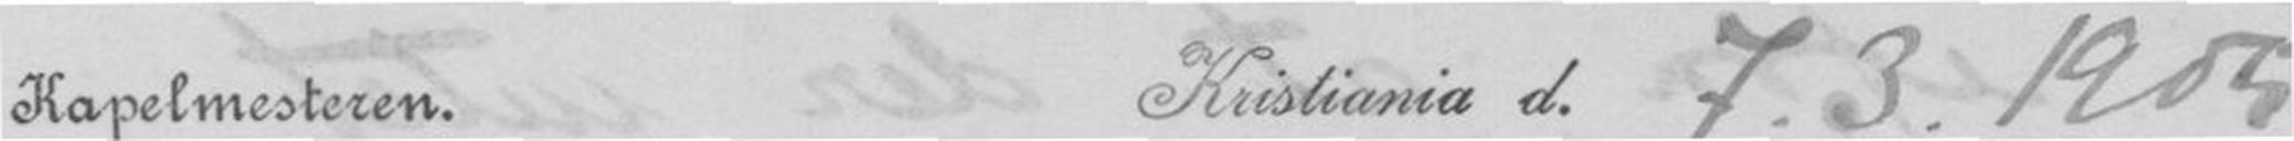Kapelmesteren. Kristiania d. 7.3.1905
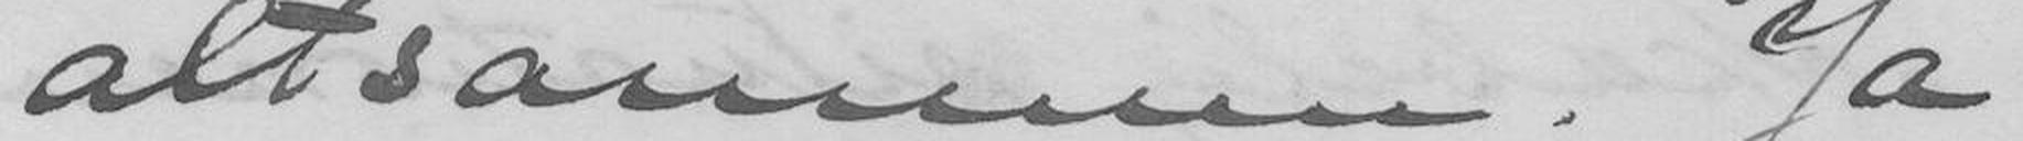altsammen. Ja
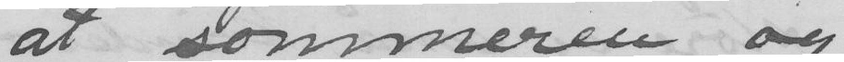at sommeren og
